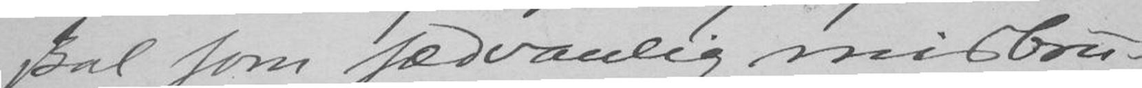skal som sædvanlig misbru-
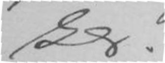kes.
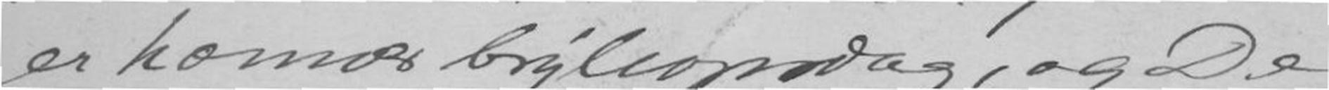er hænnes bryllopsdag, og De
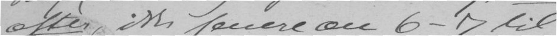æfter, ikke senere æn 6-7 til
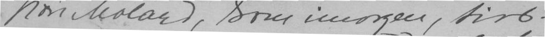Kære Molard, kom imorgen, tirs-
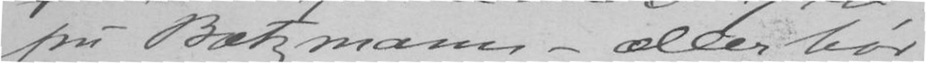fru Bætzmann - æller hør
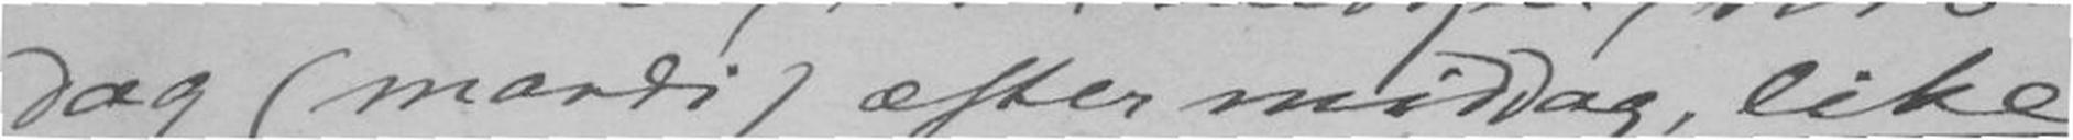dag (mardi) æfter middag, like
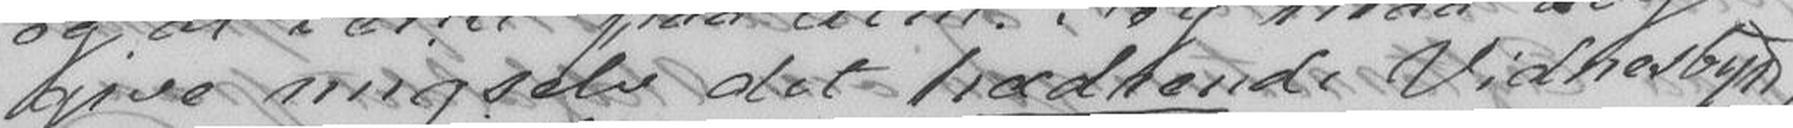give mig selv det hædrende Vidnesbyrd
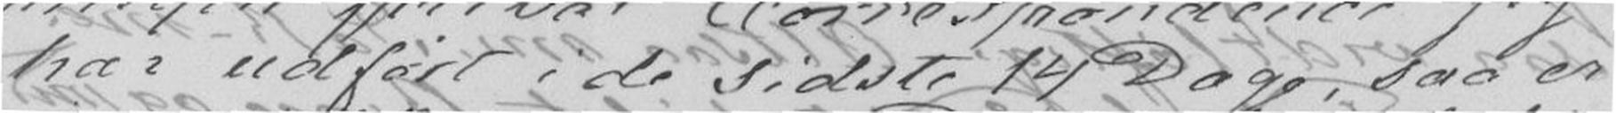har udført i de sidste 14 Dage, saa er
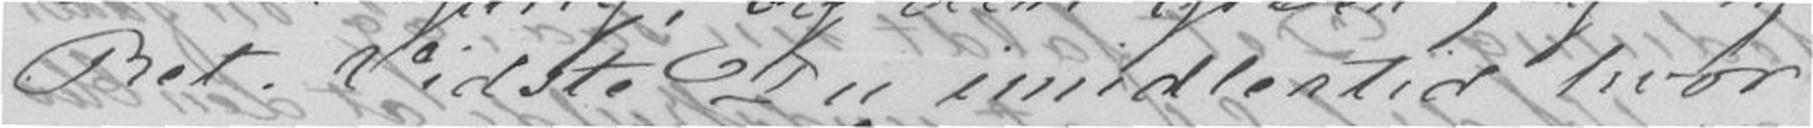Ret. Vidste Du imidlertid hvor
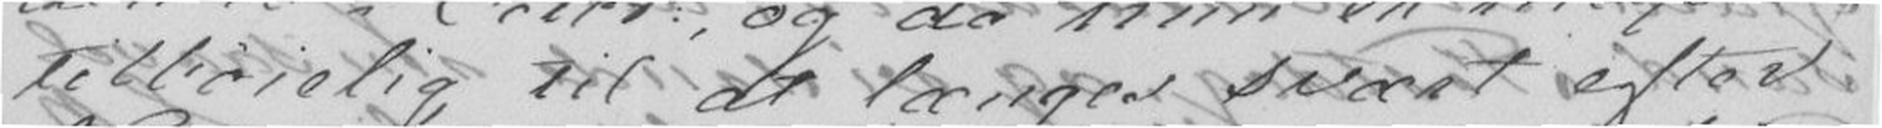tilbøielig til at længes svært efter
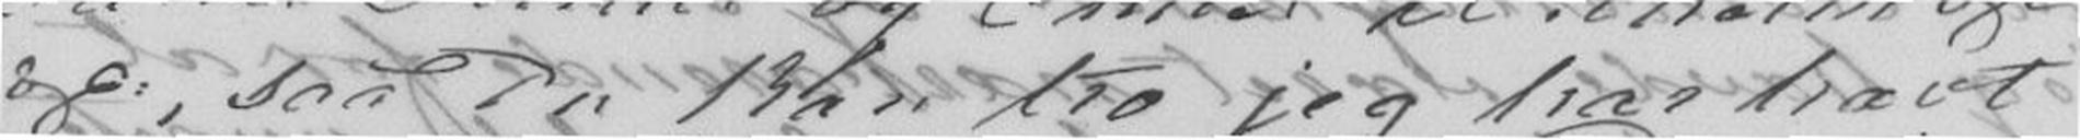ogc, saa Du kan tro jeg har havt
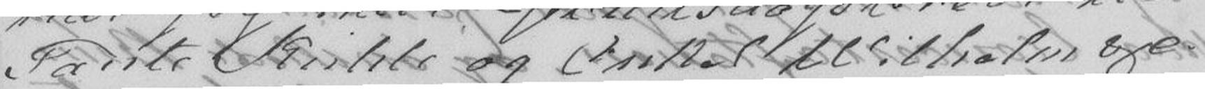Tante Kühle og Onkel Wilhelm ogc
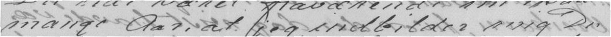mange Aar, at jeg indbilder mig Du
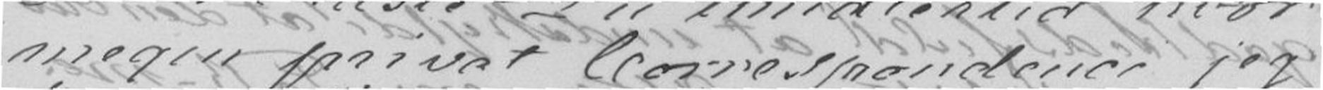megen privat Correspondance jeg
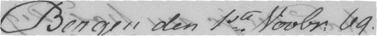Bergen den 1ste Novbr. 69.
